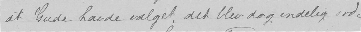at Gude havde valget, det blev dog endelig ord
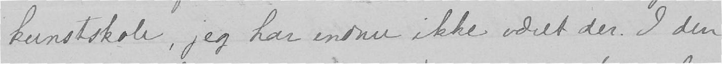kunstskole, jeg har endnu ikke været der. I den
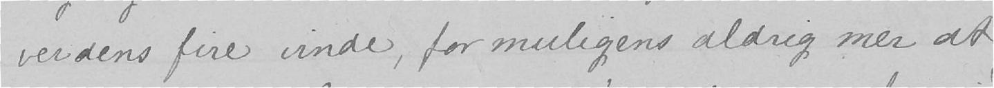verdens fire vinde, for muligens aldrig mer at
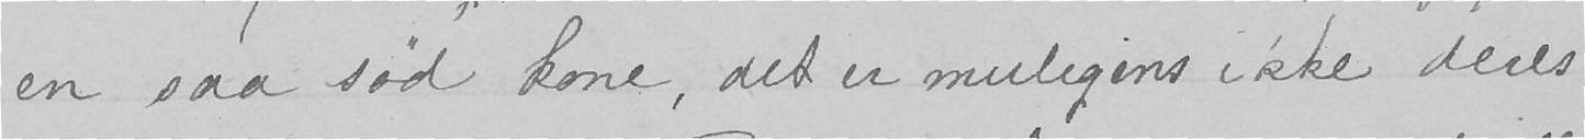en saa sød kone, det er muligens ikke deres
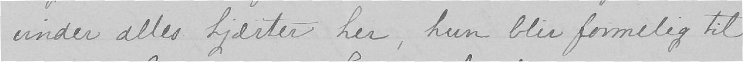vinder alles hjærter her, hun blir formelig til
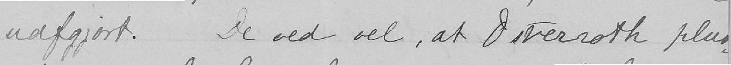uafgjort. De ved vel, at Osterroth plud-
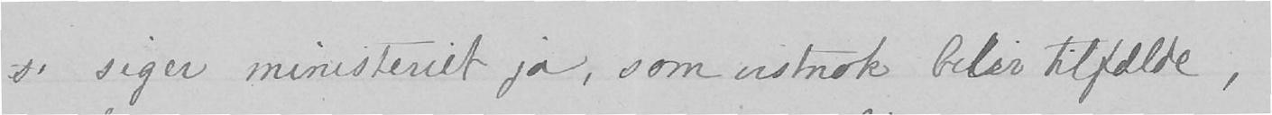s siger ministeriet ja, som vistnok blir tilfælde,
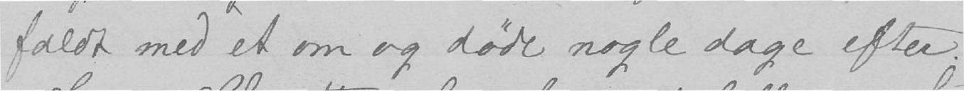faldt med et om og døde nogle dager efter.
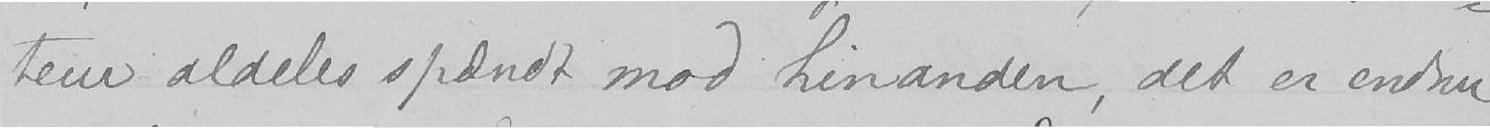teur aldeles spændt mod hinanden, det er endnu
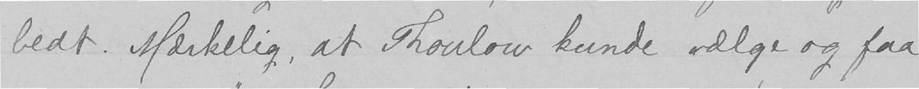bedt. Mærkelig, at Thoulow kunde vælge og faa
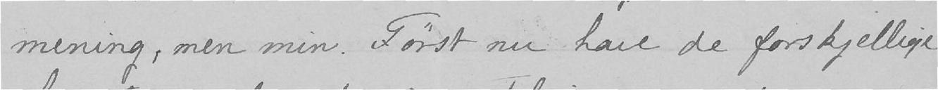mening, men min. Først nu have de forskjellige
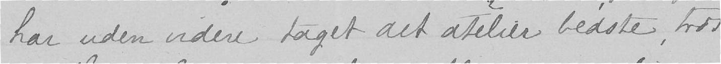har uden videre taget det atelier bedste trods
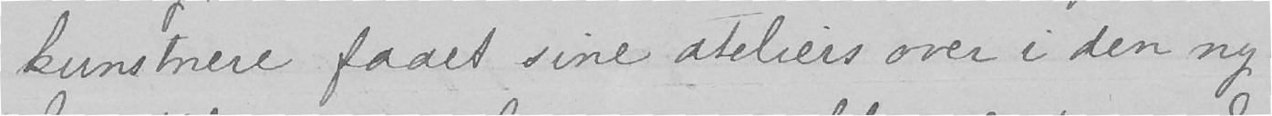kunstnere faaet sine ateliers over i den ny
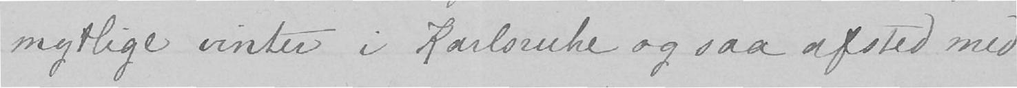mytlige vinter i Karlsruhe og saa afsted med
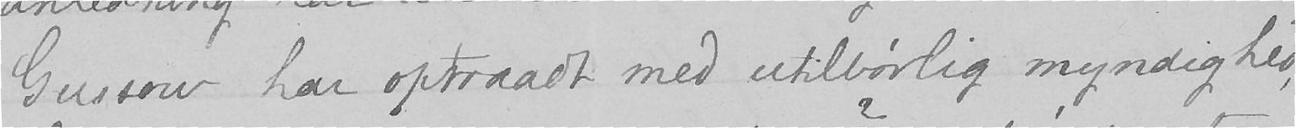Gussow har optraadt med utilbørlig myndighed,
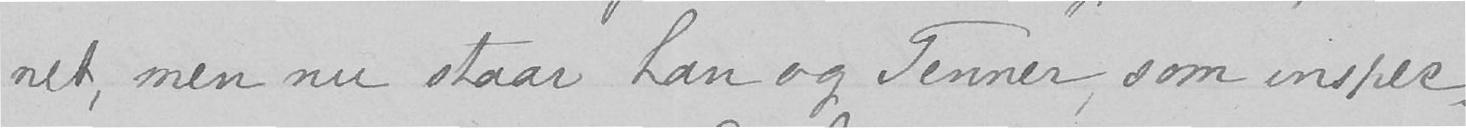net, men nu staar han og Tenner, som inspec
